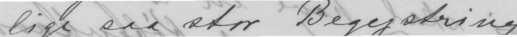lige saa stor Begejstring
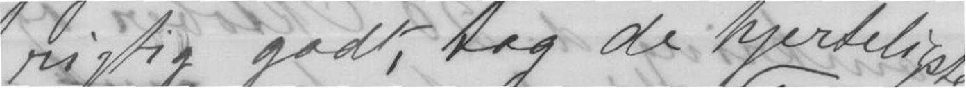rigtig godt, dog de hjerteligste
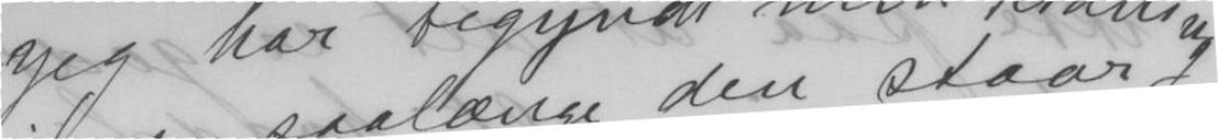Jeg har begyndt min Ridning
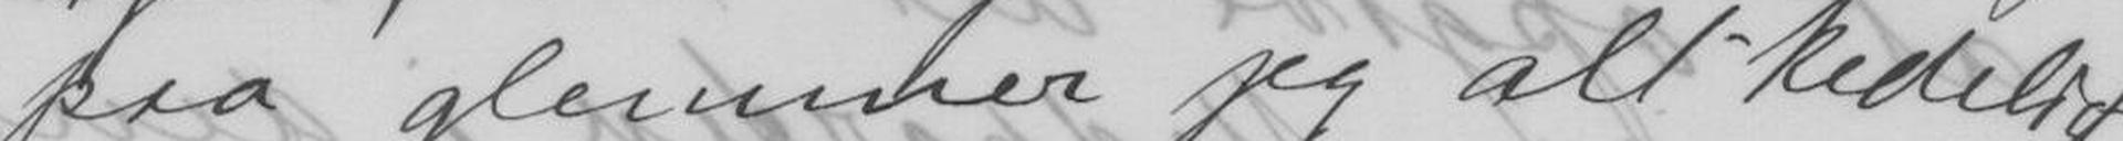paa glemmer jeg alt kedeligt
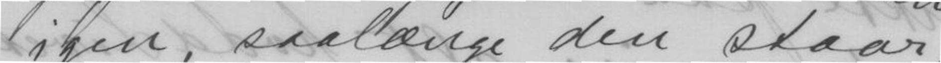igen, saalænge den staar
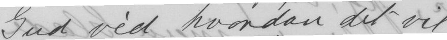Gud véd hvordan det vil
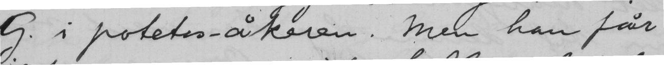G. i poteter-åkeren. Men han får
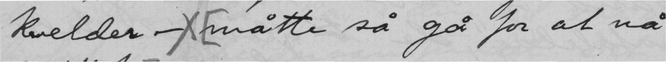kvelder - x måtte så gå for at nå
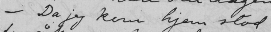- Da jeg kom hjem stod
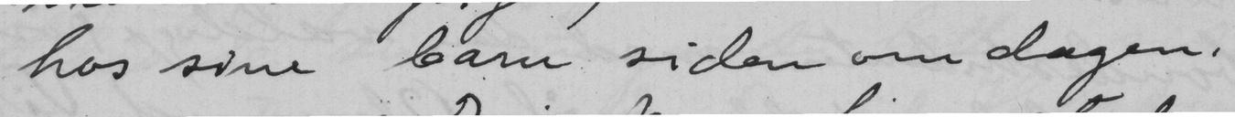hos sine barn siden om dagen.
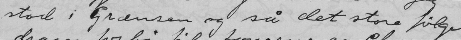stod i Grænsen og så det store følge
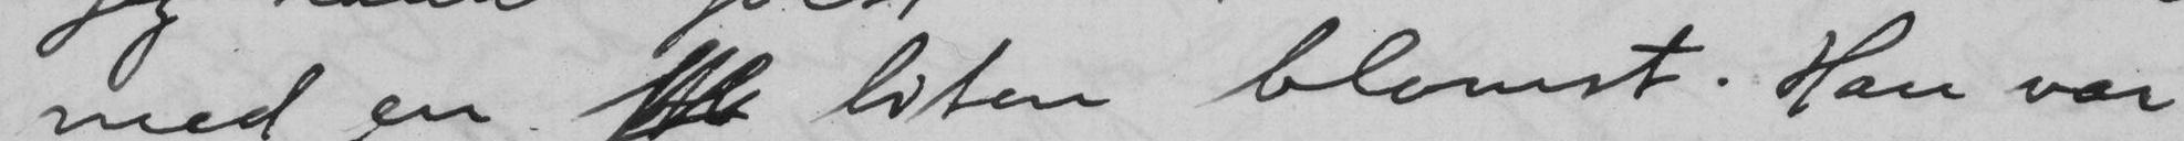med en  liten blomst. Han var
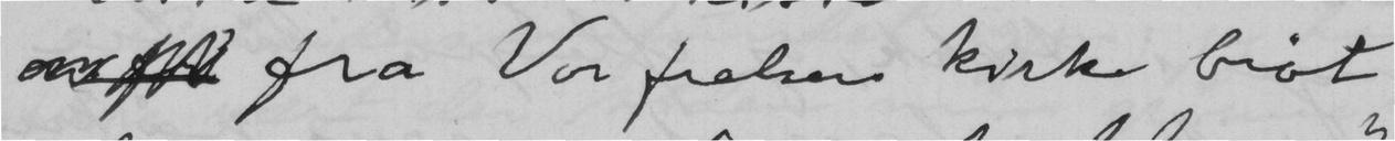fra Vor frelser kirke brøt
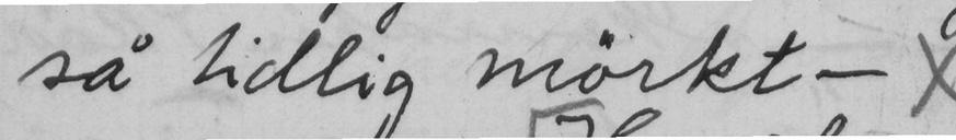så tidlig mørkt - x
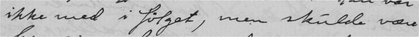ikke med i følget, men skulde være
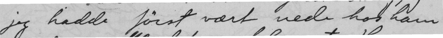jeg hadde først vært nede hos ham
